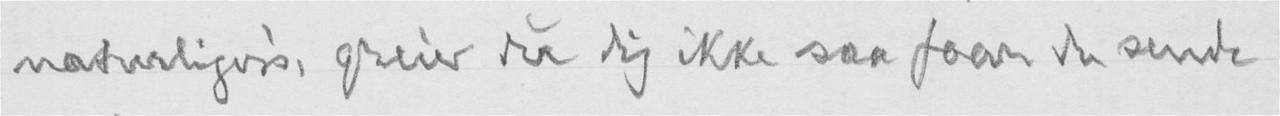naturligvis, greier du dig ikke saa faar du sende
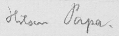Hilsen Papa.
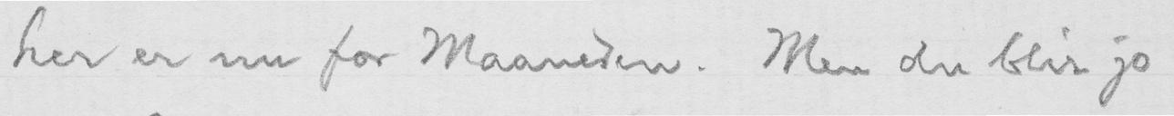her er nu for Maaneden. Men du blir jo
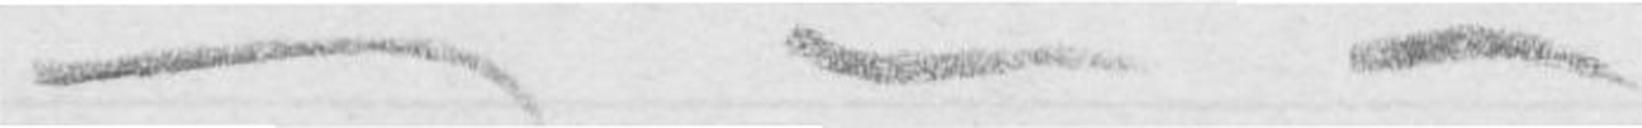- - -
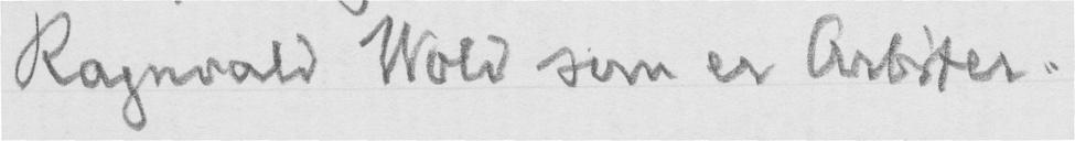Ragnvald Wold som er Arbiter.
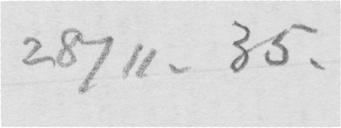28/11. 35.
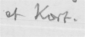et Kort.
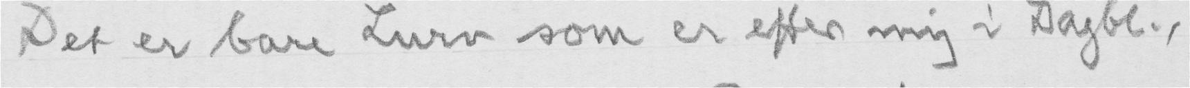Det er bare Lurv som er efter mig i Dagbl.,
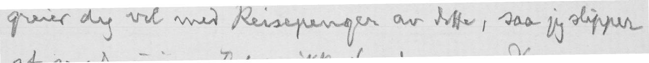greier dig vel med Reisepenger av dette, saa jeg slipper
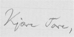Kjære Tore,
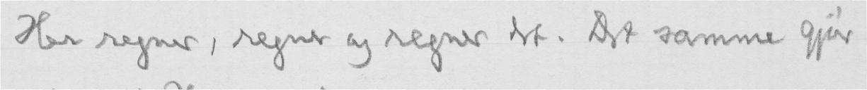Her regner, regner og regner det. Det samme gjør
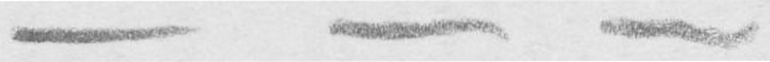- - -
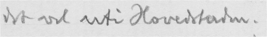det vel uti Hovedstaden.
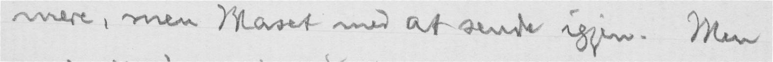mere, men Maset med at sende igjen. Men
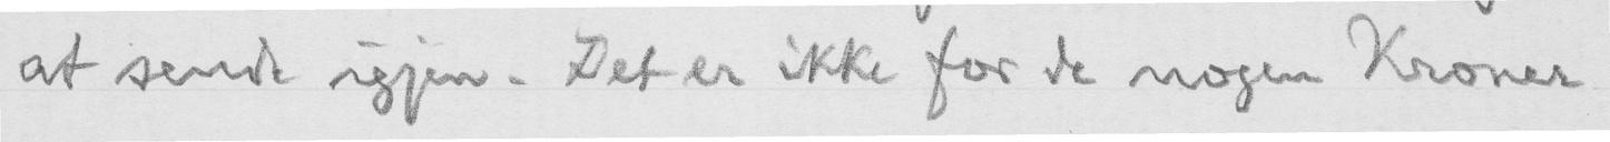at sende igjen. Det er ikke for de nogen Kroner
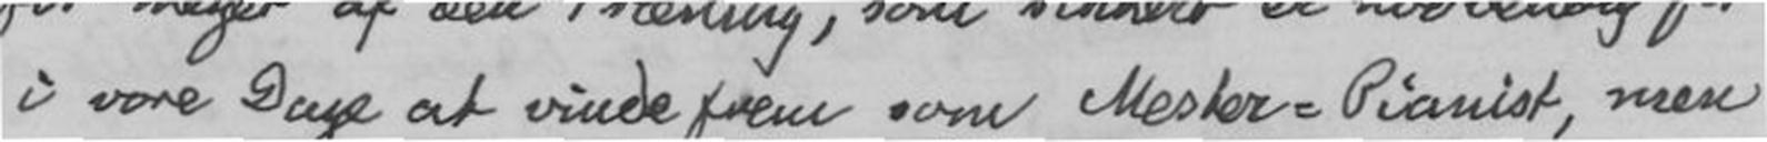'i vore Dage at vinde frem som Mester=Pianist, men
